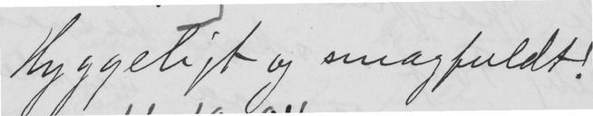Hyggeligt og smagfuldt!
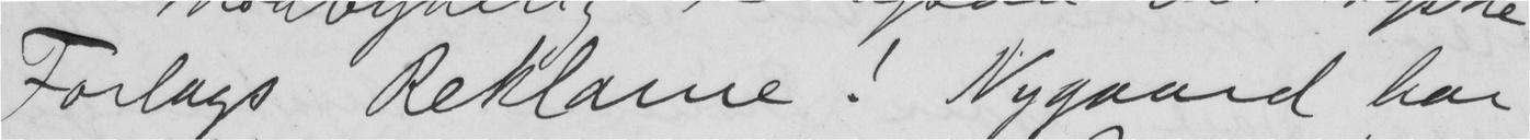Forlags Reklame! Nygaard har
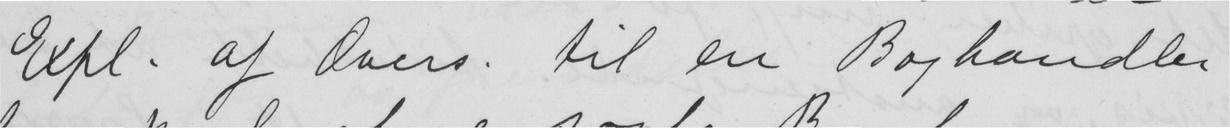Expl. af Overs. til en Boghandler
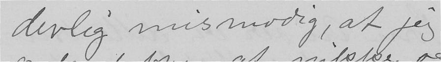derlig mismodig, at jeg
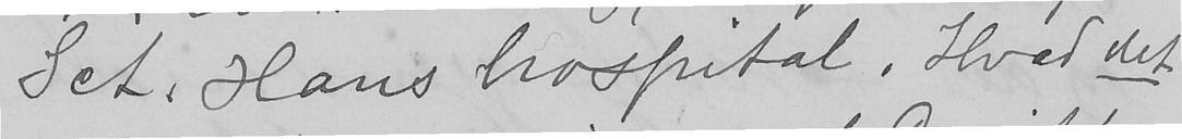Sct. Hans hospital. Hvad det
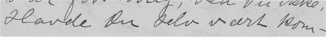Havde Du selv vært kom-
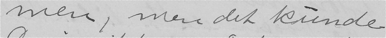men, men det kunde
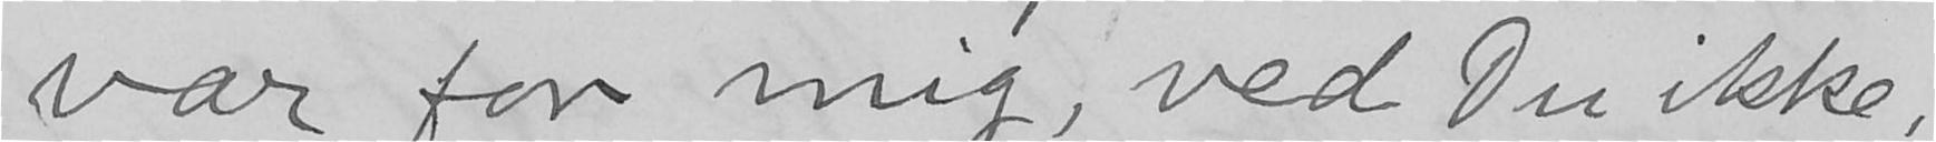var for mig, ved Du ikke.
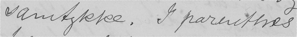samtykke. I parenthes
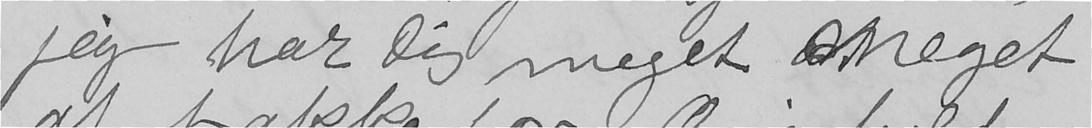jeg har Dig meget meget
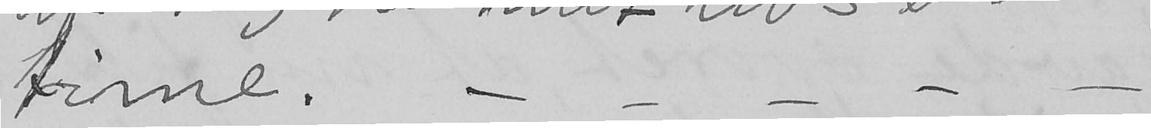time. - - - - -
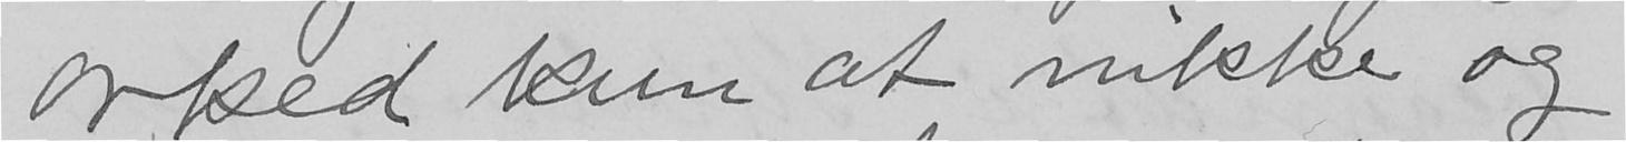orked kun at nikke og
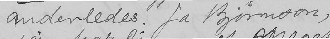anderledes. Ja Bjørnson,
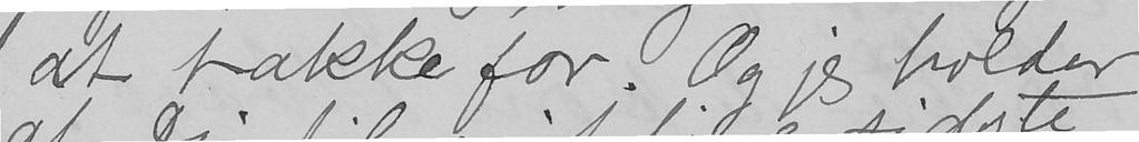at takke for. Og jeg holder
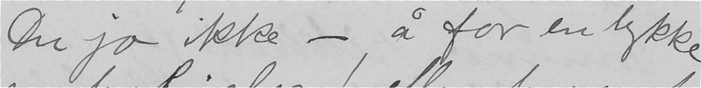Du jo ikke - å for en lykke
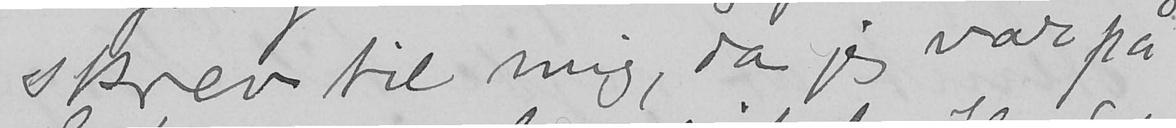skrev til mig, da jeg var på
